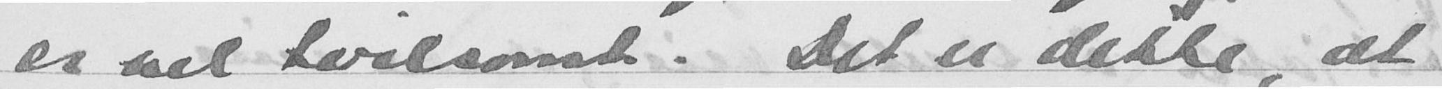er vel tvilsomt. Det er dette, at
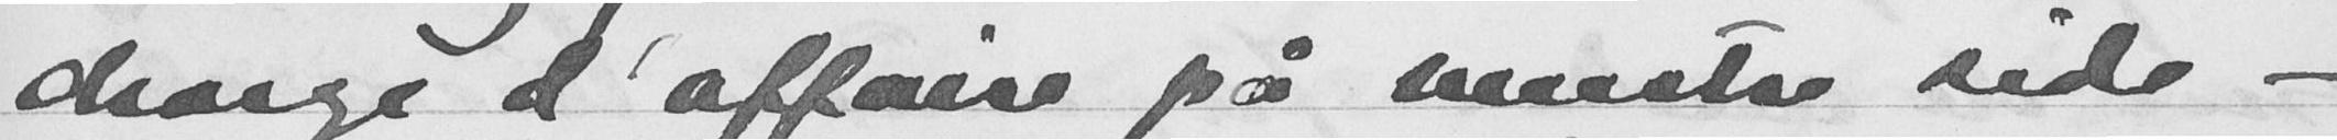charge d'affairs på venstre side -
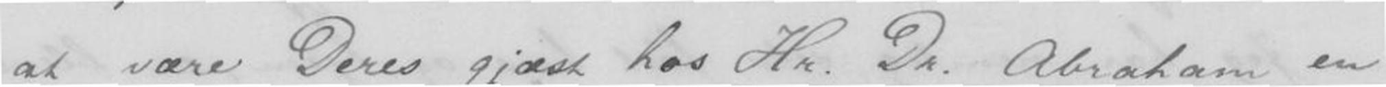at være Deres gjæst hos Hr. Dr. Abraham en
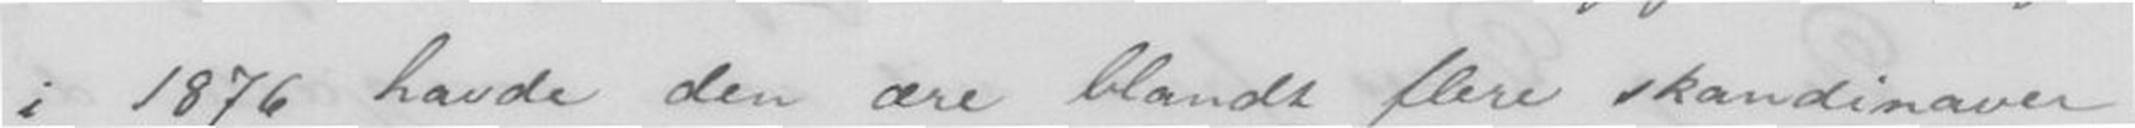i 1876 havde den ære blandt flere skandinaver
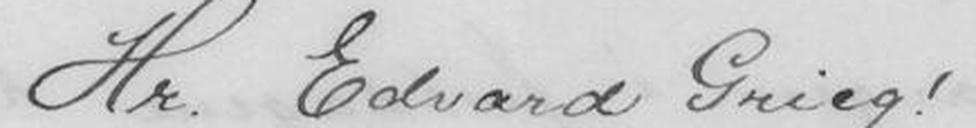Hr Edvard Grieg!
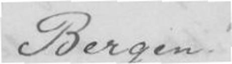Bergen!
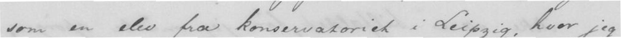som en elev fra konservatoriet i Leipzig, hvor jeg
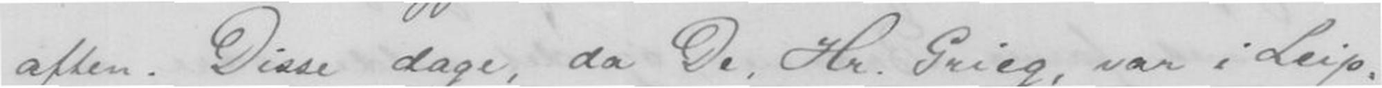aften. Disse dage, da De, Hr. Grieg, var i Leip-
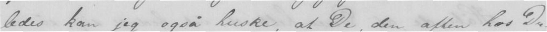ledes kan jeg også huske, at De, den aften hos Dr.
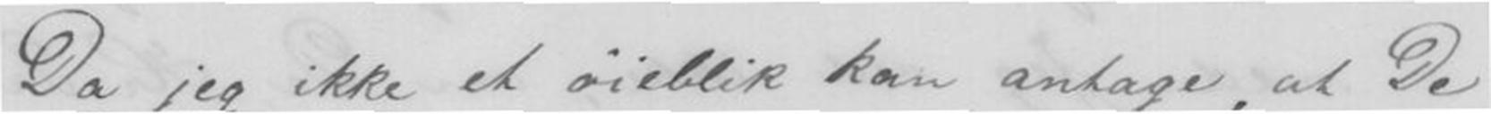Da jeg ikke et øieblik kan antage, at De
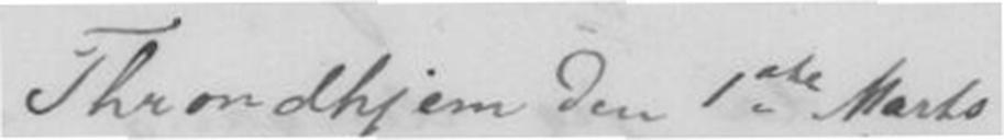Trondhjem den 1ste Marts
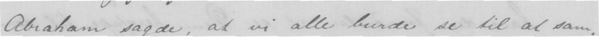Abraham sagde, at vi alle burde se til at sam-
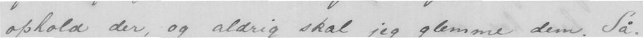ophold der, og aldrig skal jeg glemme dem, Så-
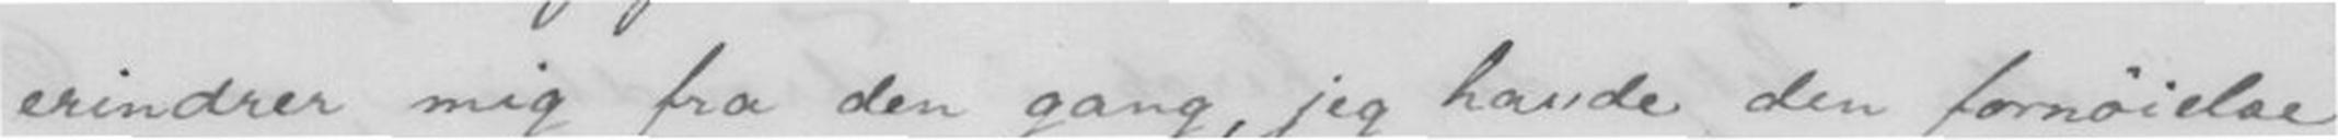erindrer mig fra den gang, jeg havde den fornøielse
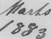1883
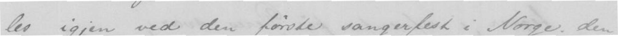les igjen ved den første sangerfest i Norge; den
